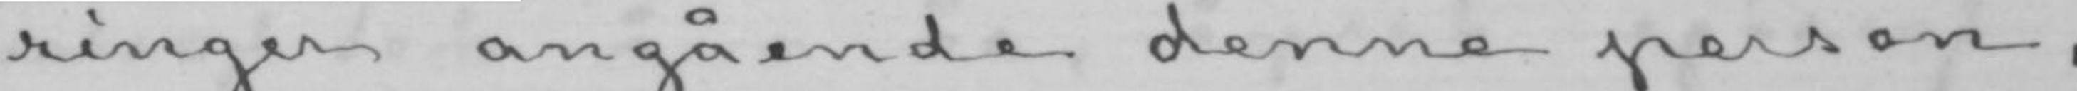ringer angående denne person,
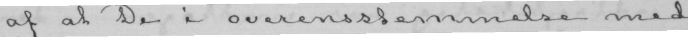af at De i overensstemmelse med
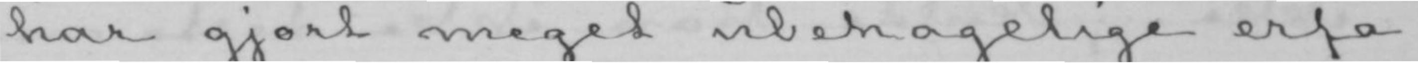har gjort meget ubehagelige erfa-
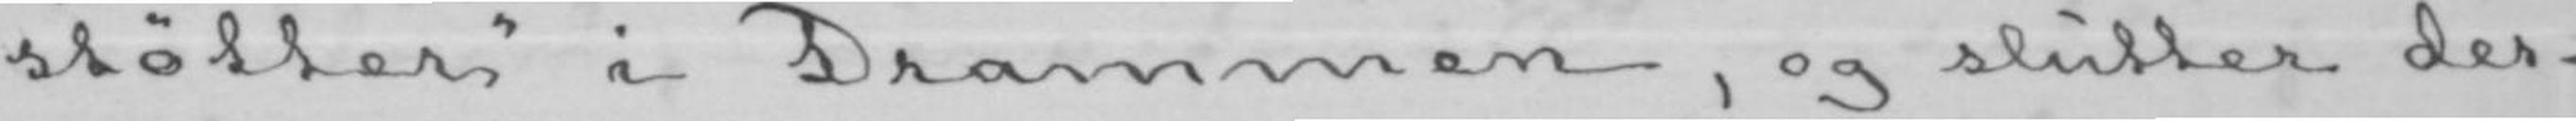støtter» i Drammen, og slutter der-
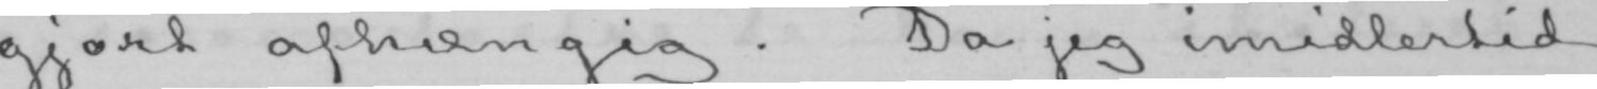gjort afhængig. Da jeg imidlertid
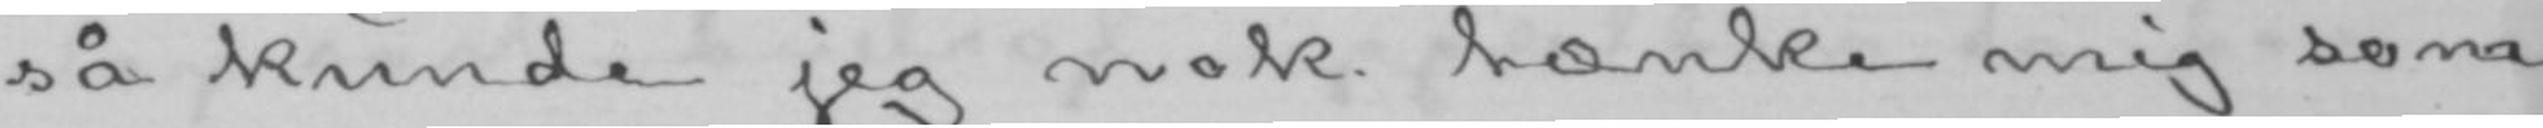så kunde jeg nok tænke mig som
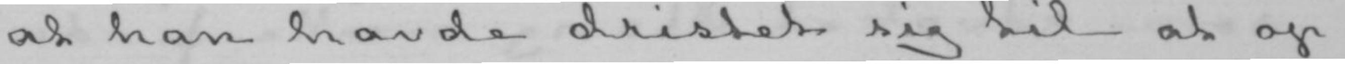at han havde dristet sig til at op-
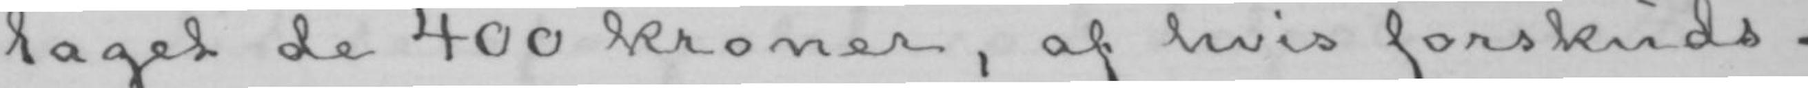taget de 400 kroner, af hvis forskuds-
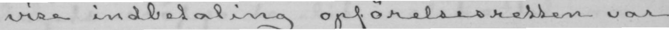vise indbetaling opførelsesretten var
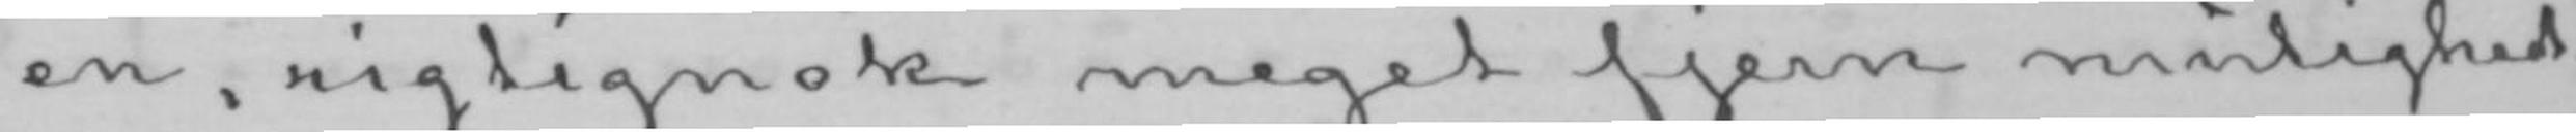en, rigtignok meget fjern mulighed,
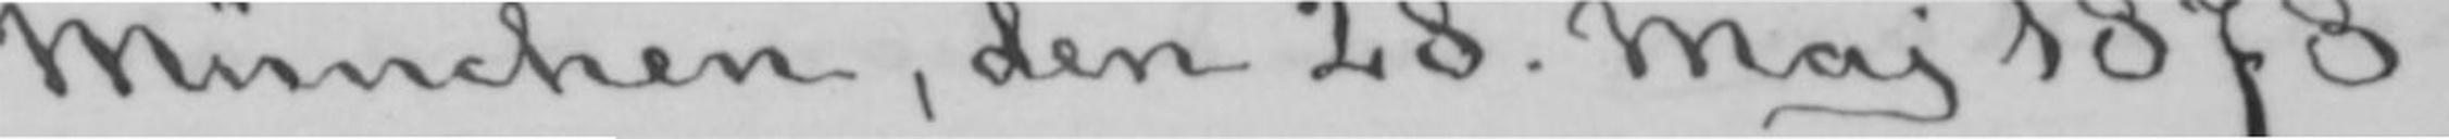München, den 28. Maj 1878.
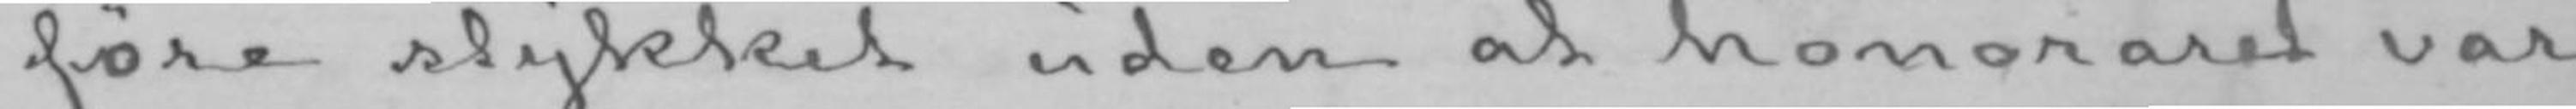føre stykket uden at honoraret var
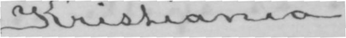Kristiania.
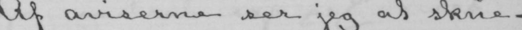Af aviserne ser jeg at skue-
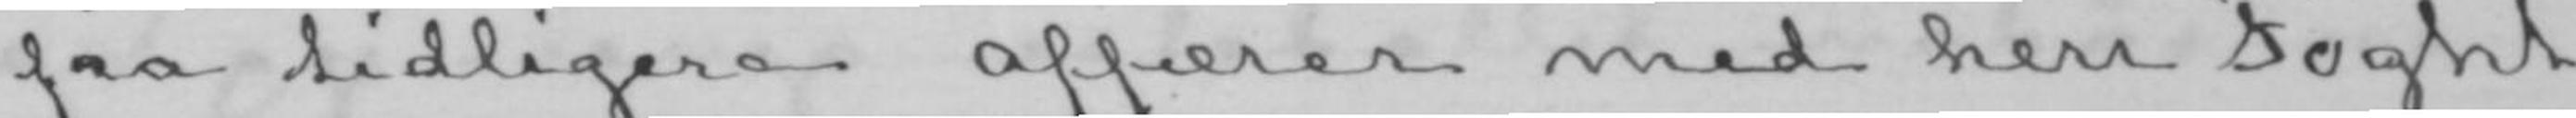fra tidligere affærer med herr Foght
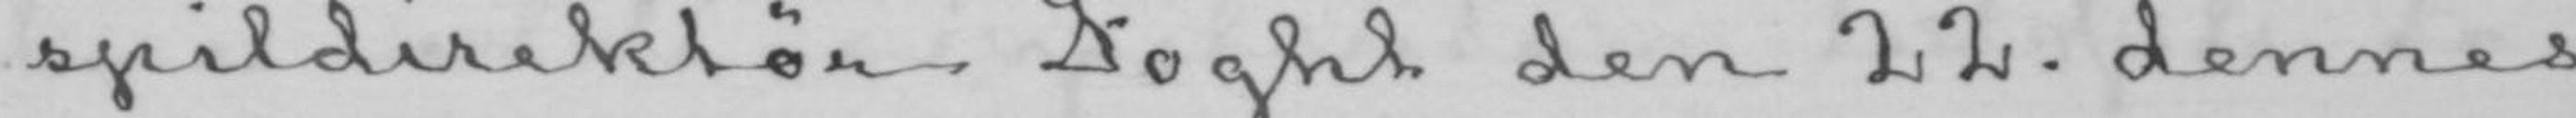spildirektør Foght den 22. dennes
